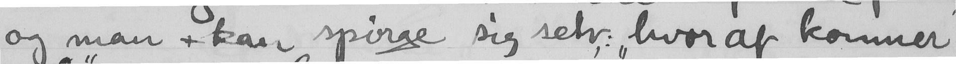og man kan spørge sig selv,: "hvoraf kommer
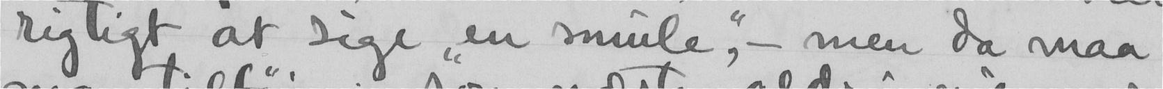rigtigt at sige "en smule", - men da maa
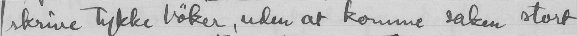skrive tykke bøker, uden at komme saken stort
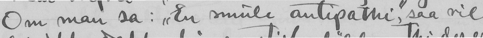Om man sa. "En smule antipathi", saa vil
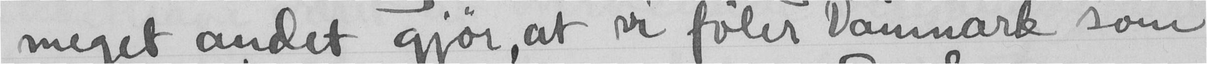meget andet gjør, at vi føler Danmark som
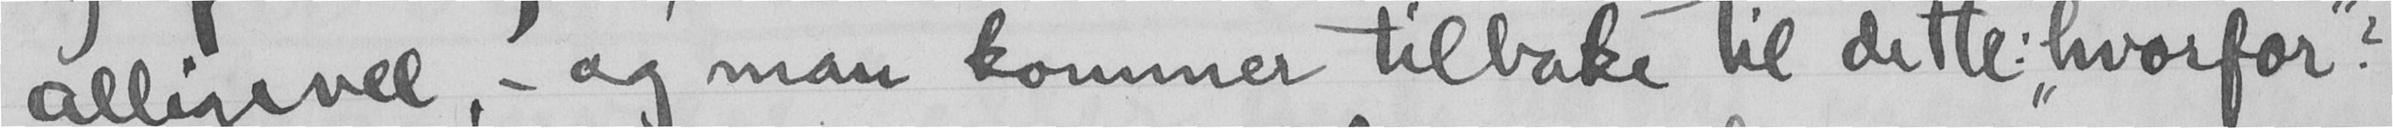alligevel, - og man kommer tilbake til dette: "hvorfor"?
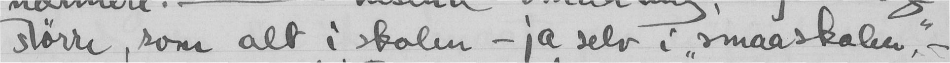større, som alt i skolen - ja selv i "smaaskolen", -
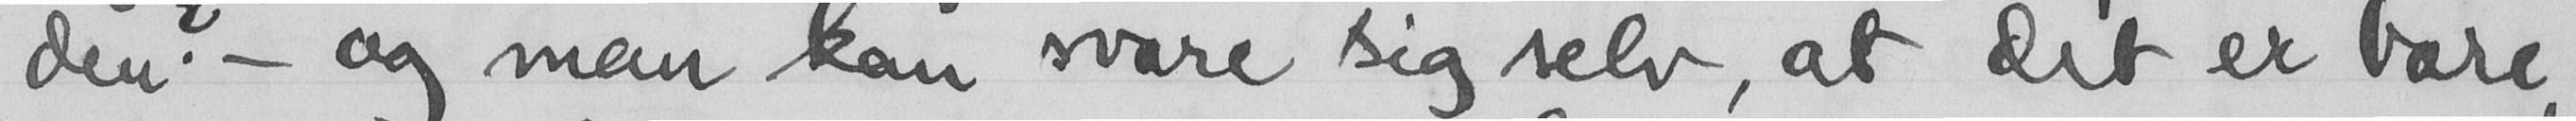den? - og man kan svare sig selv, at det er bare
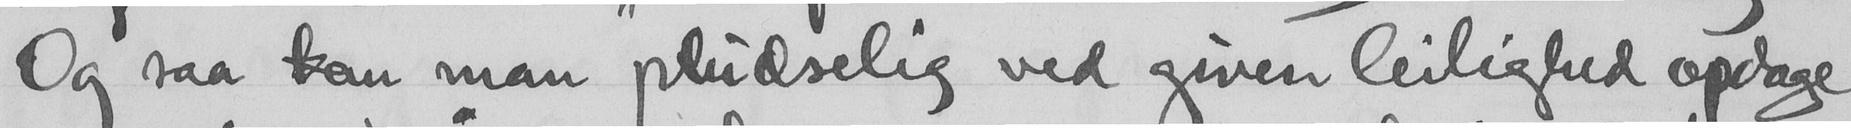Og saa kan man pludselig ved given leilighed opdage
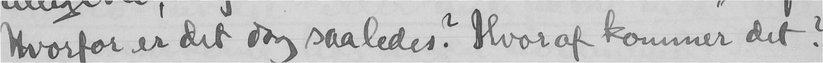Hvorfor er det dog saaledes? Hvoraf kommer det?
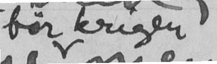før krigen
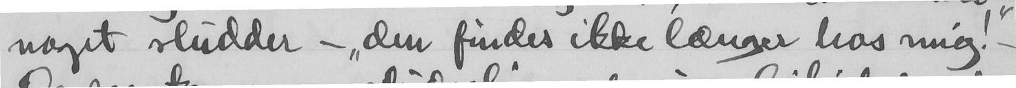noget sludder - "den findes ikke længer hos mig!"
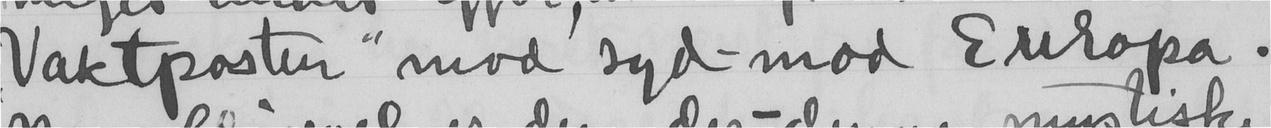"Vaktposten" mod syd - mod Europa.
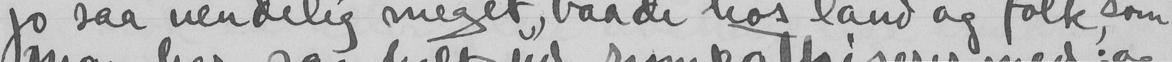jo saa uendelig meget, baade hos land og folk som
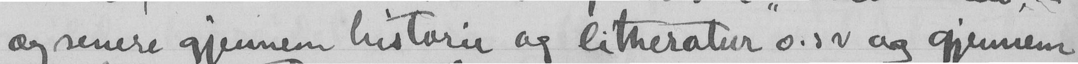og senere gjennem historie og litheratur o.s.v og gjennem
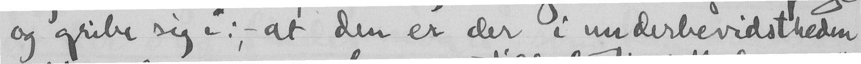og gribe sig i: - at den er der i underbevidstheden
